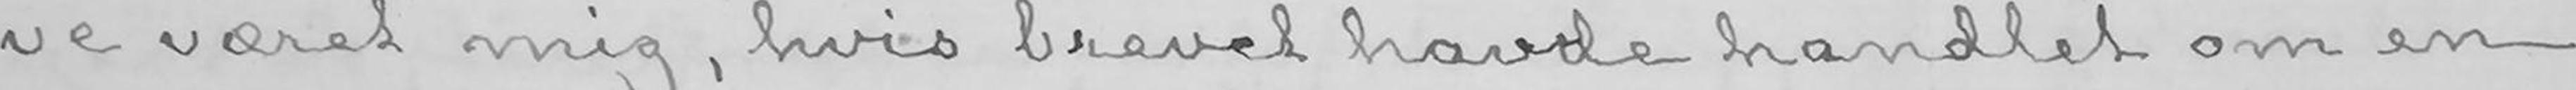ve været mig, hvis brevet havde handlet om en
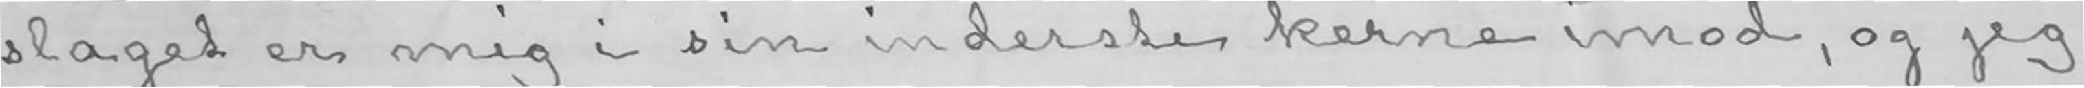slaget er mig i sin inderste kerne imod, og jeg
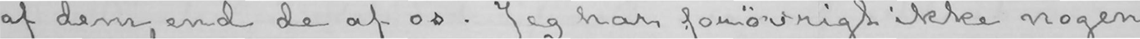af dem, end de af os. Jeg har forøvrigt ikke nogen
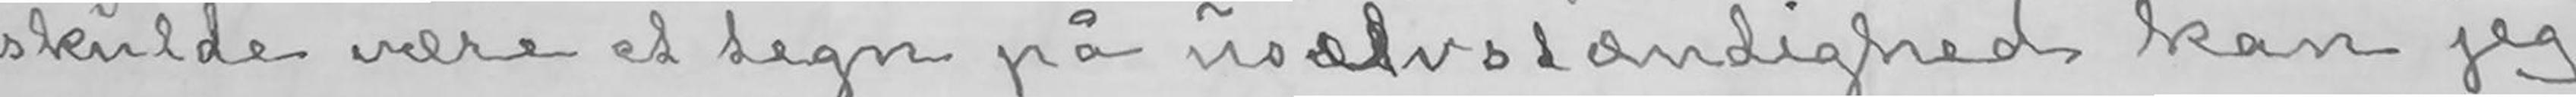skulde være et tegn på uselvstændighed kan jeg
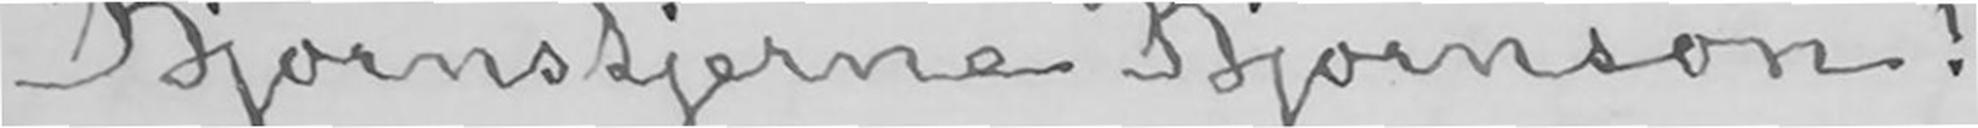Bjørnstjerne Bjørnson!
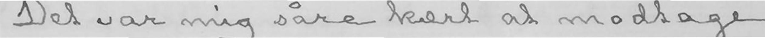Det var mig såre kært at modtage
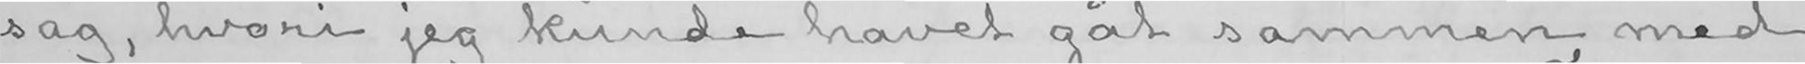sag, hvori jeg kunde havet gåt sammen med
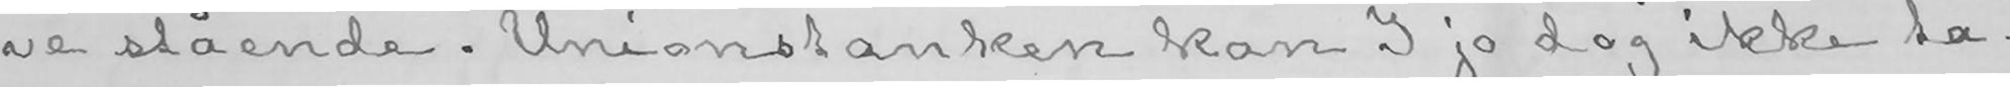ve stående. Unionstanken kan I jo dog ikke ta-
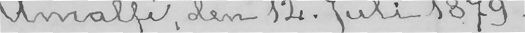Amalfi, den 12. Juli 1879.
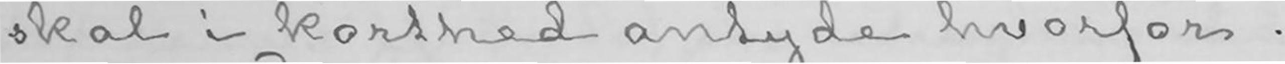skal i korthed antyde hvorfor.
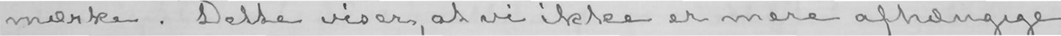mærke. Dette viser, at vi ikke er mere afhængige
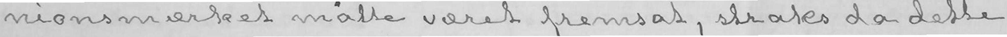nionsmærket måtte været fremsat, straks da dette
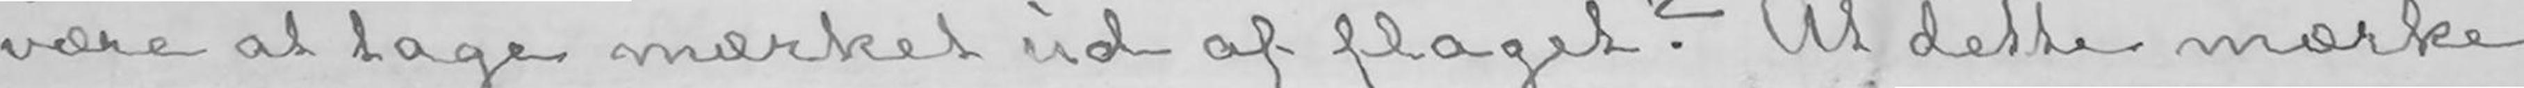være at tage mærket ud af flaget? At dette mærke
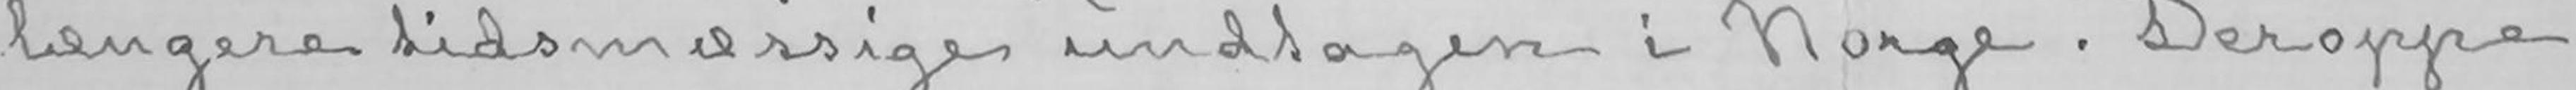længere tidsmæssige undtagen i Norge. Deroppe
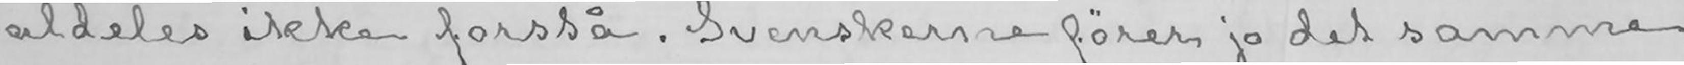aldeles ikke forstå. Svenskerne fører jo det samme
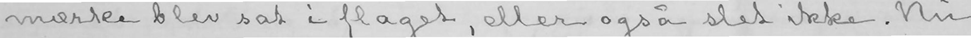mærke blev sat i flaget, eller også slet ikke. Nu
